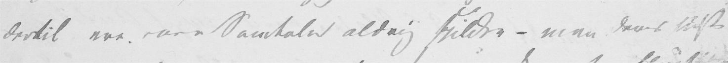dertil ere vore Samtaler aldrig spildte – men Deres sidste
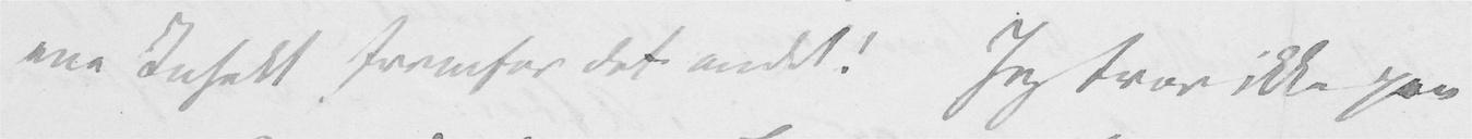ene Insekt fremfor det andet! Jeg tror ikke paa
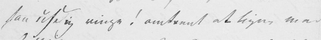saa usigelig ringe! omtrent at ligne med
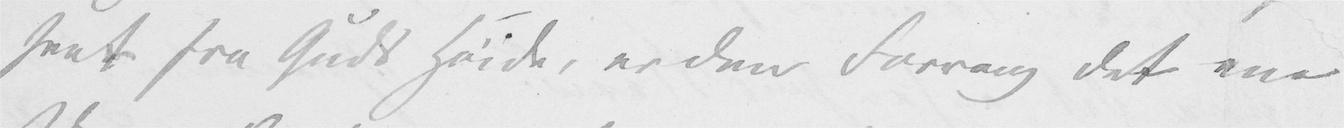seet fra Guds Høide, er den Forrang det ene
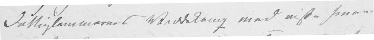Fattiglemmernes Veddekamp med visse smaa
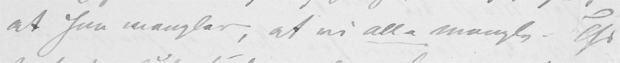at han mangler, at vi alle mangle. Thi
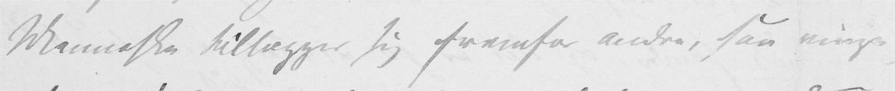Menneske tillægger sig fremfor andre, saa ringe
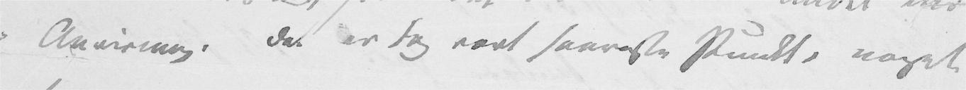Anvisning. Det er dog vort saareste Punkt, noget
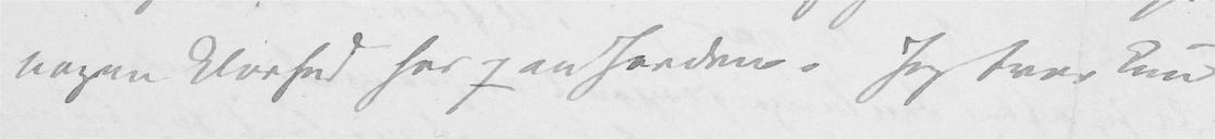nogen Klarhed her paa Jorden. Jeg tror kun
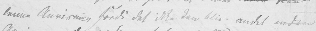denne Anvisning, fordi det ikke kan blive andet end en
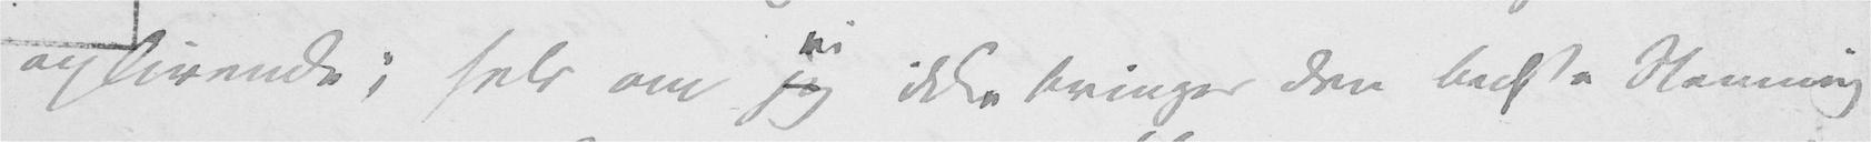oplivende; selv om jeg vi ikke bringer den bedste Stemning
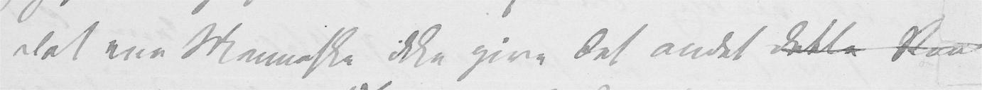det ene Menneske ikke give det andet dette Raad
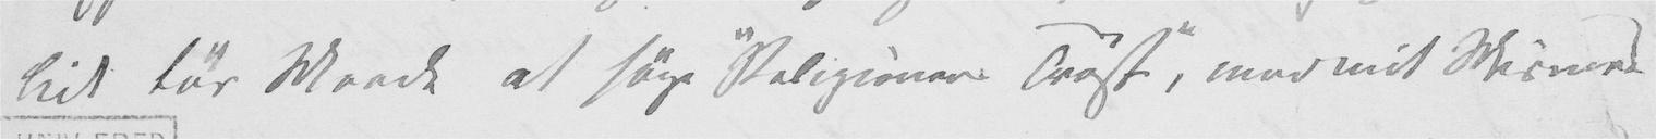lidt tør Maade at søge "Religionens Trøst", mod mit Mismod
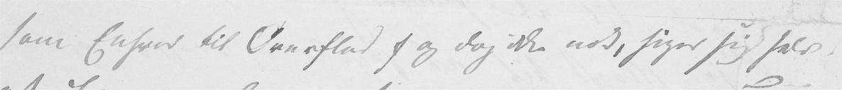som Enhver til Overflod s og dog ikke nok, siger sig selv
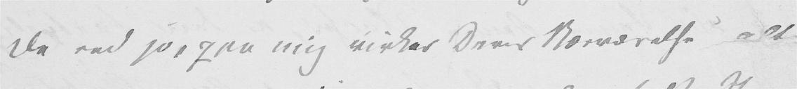De ved jo, paa mig virker Deres Nærværelse alt
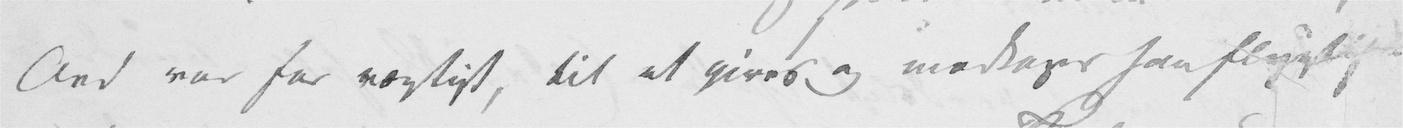Ord var for vægtigt, til at gives og modtages saa flygtigt.
